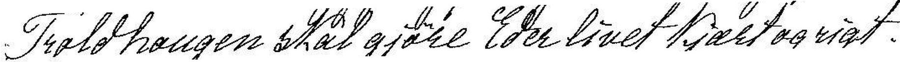Troldhaugen skal gjøre Eder livet kjært og rigt.
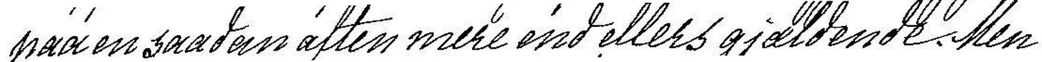paa en saadan aften mere end ellers gjældende. Men
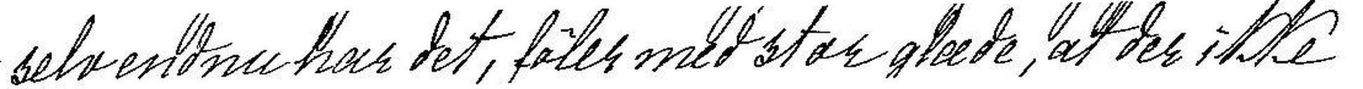selv endnu har det, føler med stor glæde, at der ikke
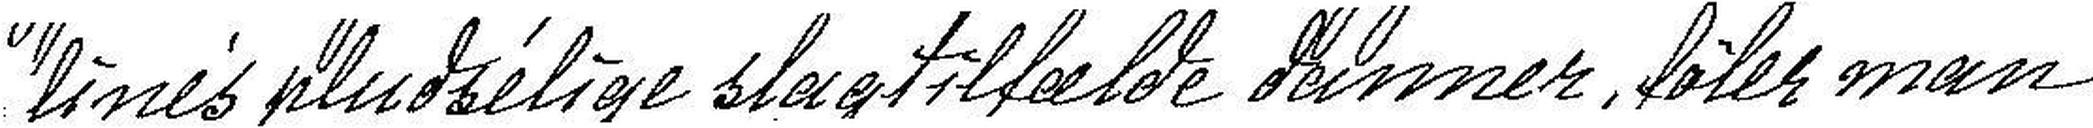line's pludselige slagtilfælde danner, føler man
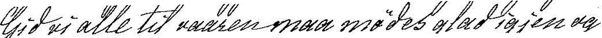Gid vi alle til vaaren maa mødes glad igjen og
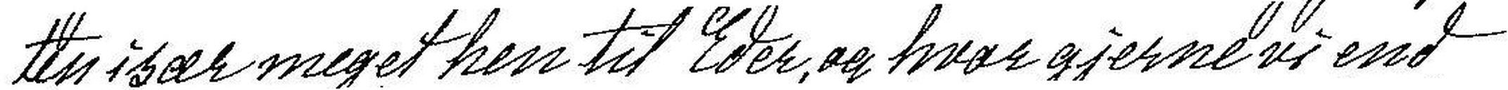ten især meget hen til Eder, og hvor gjerne vi end
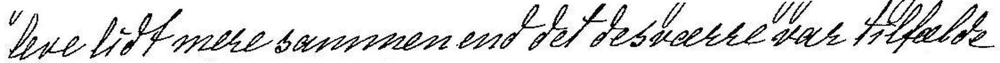leve lidt mere sammen end det desværre var tilfælde
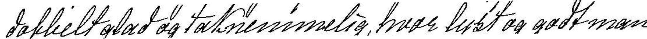dobbelt glad og taknemmelig, hvor lyst og godt man
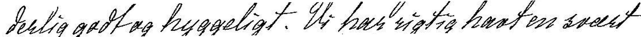derlig godt og hyggeligt. Vi har rigtig havt en svært
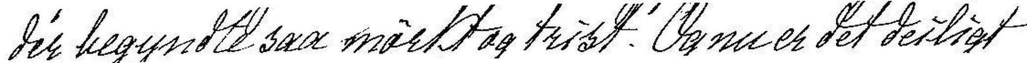der begyndte saa mørkt og trist. Og nu er det deiligt
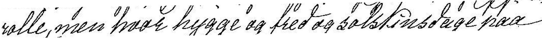rolle, men hvor hygge og fred og solskinsdage paa
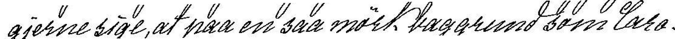gjerne sige, at paa en saa mørk baggrund som Caro-
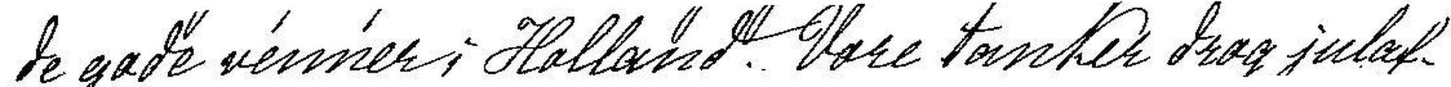de gode venner i Holland. Vore tanker drog julaf-
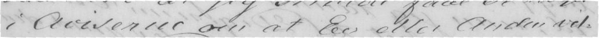i Aviserne om at En eller Anden vil-
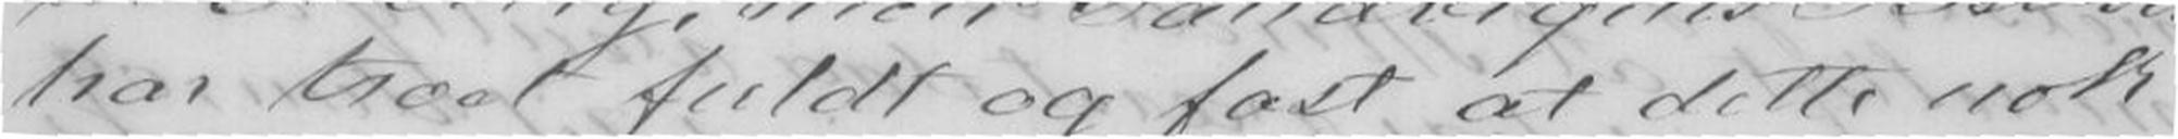har troet fuldt og fast at dette nok
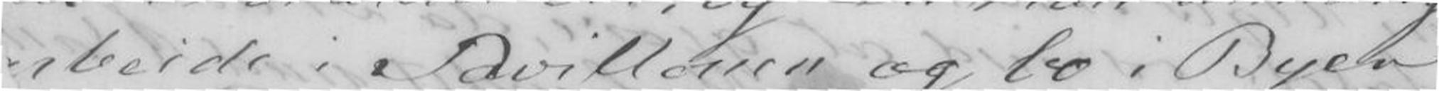rbeide i Pavillonen og bo i Byen
